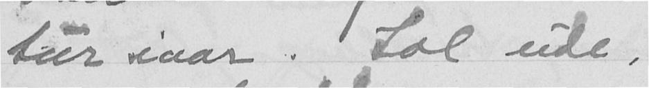tur iaar. Sol ude,
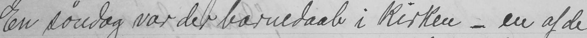En søndag var der barnedaab i kirken - en af de
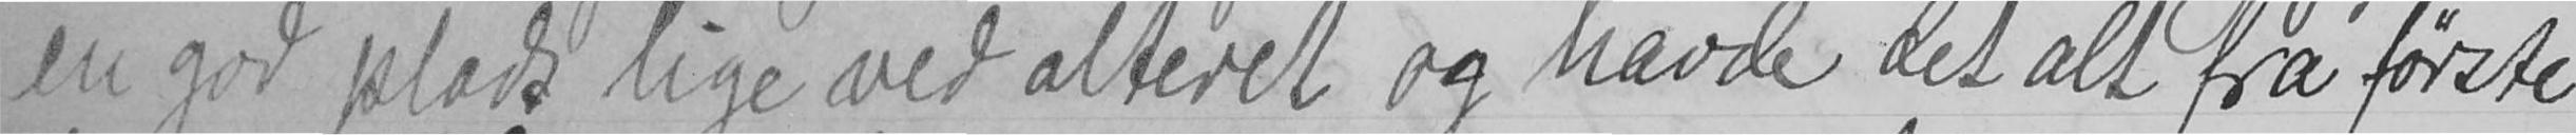en god plads lige ved alteret og havde det alt fra første
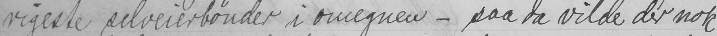rigeste selveierbønder i omegnen - saa da vilde der nok
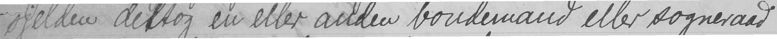sjelden deltog en eller anden bondemand eller sognemand
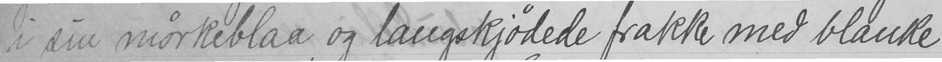i sin mørkeblaa og langskjødede frakke med blanke
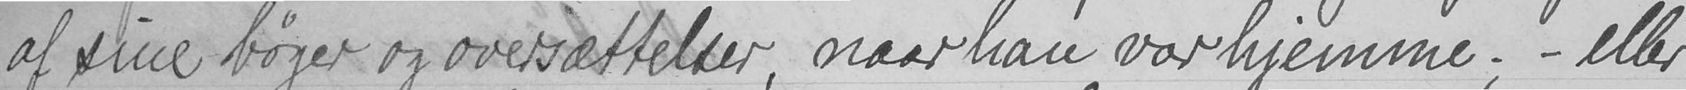af sine bøger og oversættelser, naar han var hjemme; - eller
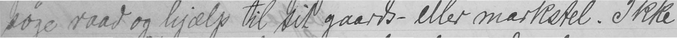søge raad og hjælp til sit gaards- eller markstel. Ikke
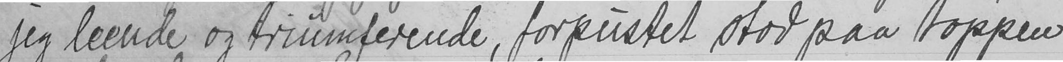jeg leende og triumferende, forpustet stod paa toppen
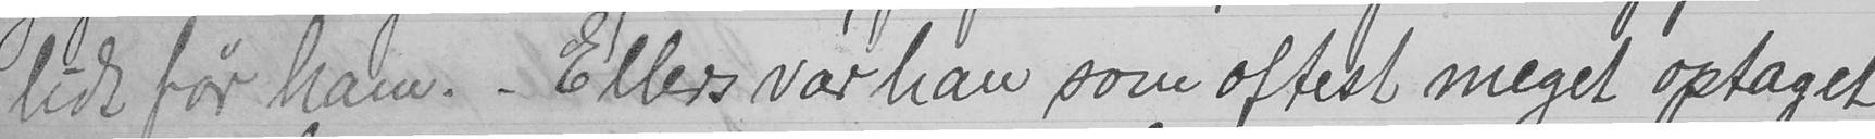lidt før ham. - Ellers var han som oftest meget optaget
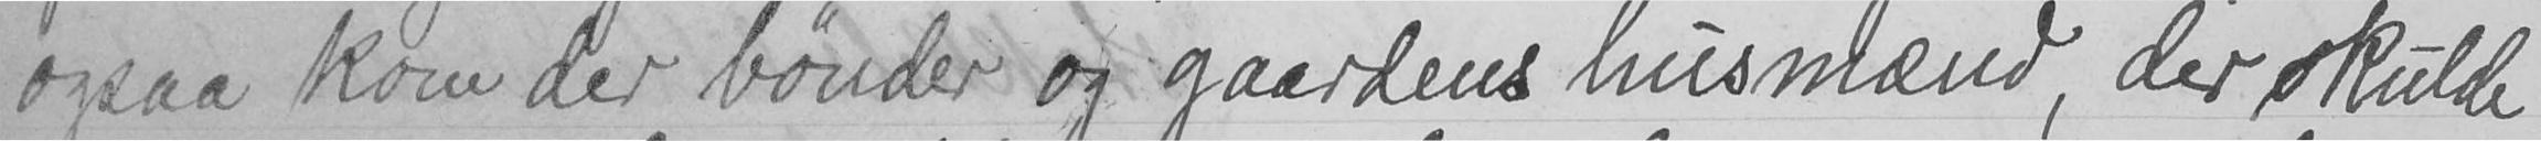ogsaa kom der bønder og gaardens husmænd, der skulde
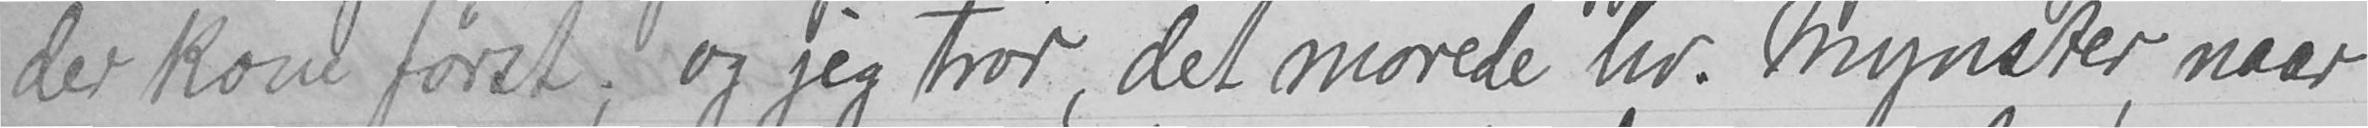der kom først; og jeg tror, det morede hr. Mynster, naar
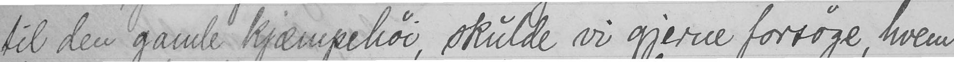til den gamle kjæmpehøi, skulde vi gjerne forsøge, hvem
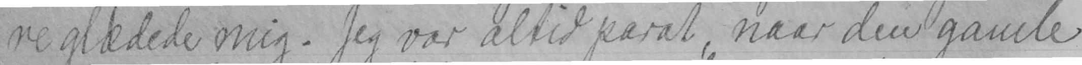re glædede mig. Jeg var altid parat naar den gamle
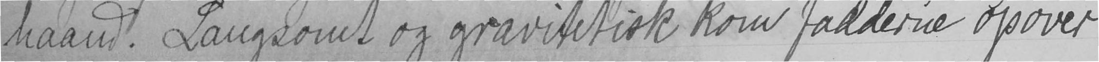haand. Langsomt og gravitetisk kom fadderne opover
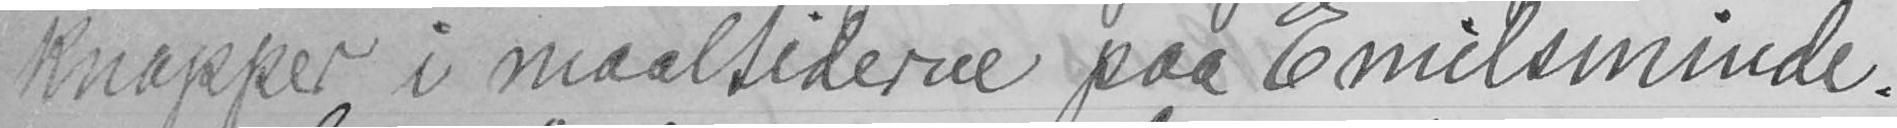knapper i maaltiderne paa Emilsminde.
